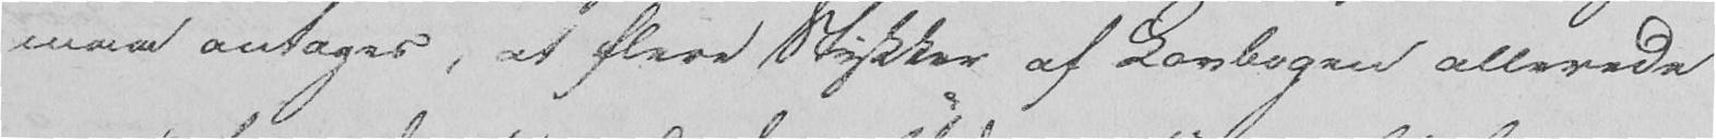maa antages, at flere Stykker af Lovbogen allerede
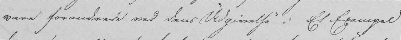vare forandrede ved dens Udgivelse. Et Exempel
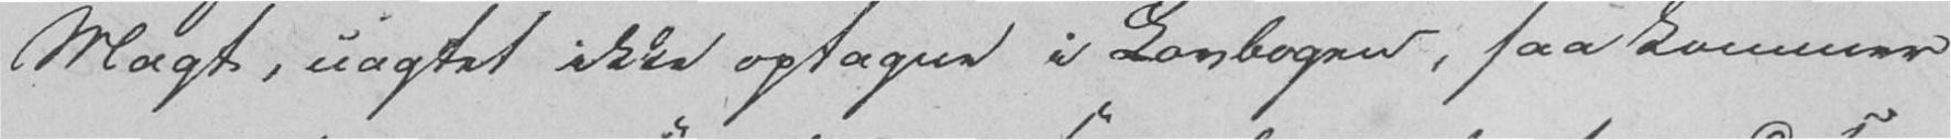Magt, uagtet ikke optagne i Lovbogen, saa kommer
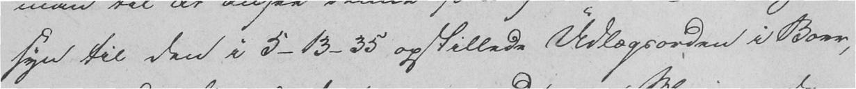syn til den i 5-13-35 opstillede Udlægsorden i Boer,
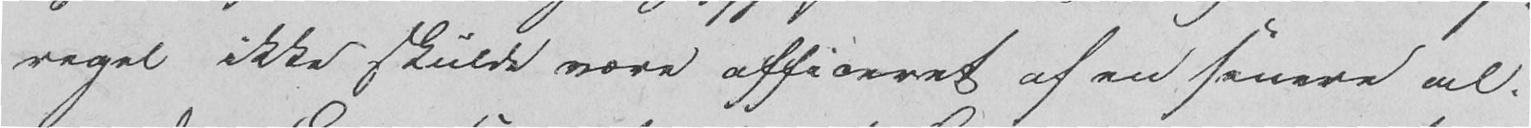regel ikke skulde være afficeret af en senere al-
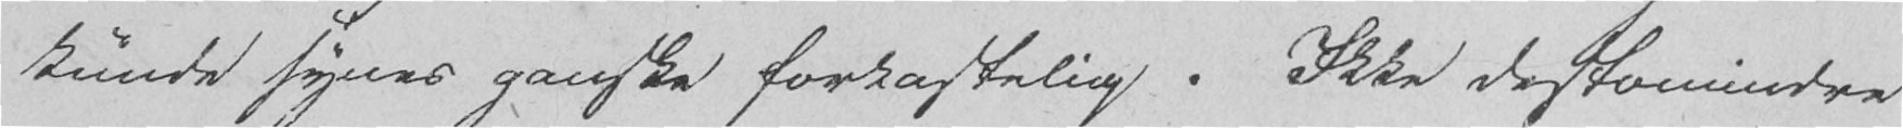kunde synes ganske forkastelig. Ikke destomindre
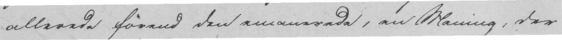allerede førend den emanerede, en Mening, der
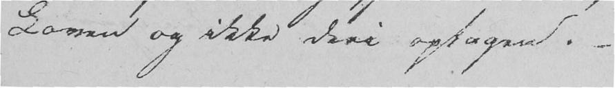Loven og ikke deri optagen. -
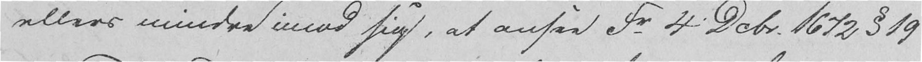ellers mindre imod sig, at ansee Fr 4. Dcbr. 1672 § 19
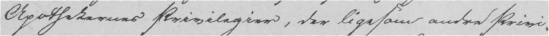Apothekernes Privilegier, der ligesom andre Privi-
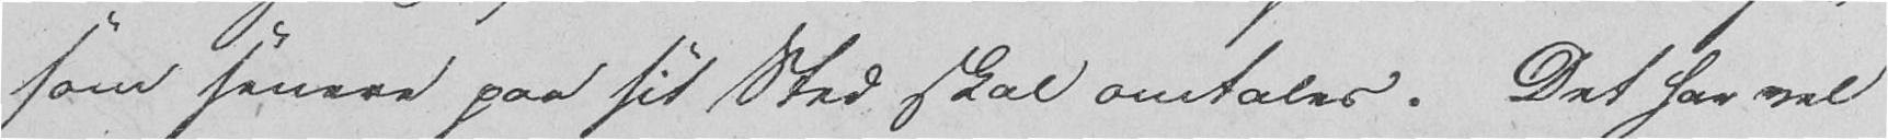som senere paa sit Sted skal omtales. Det har vel
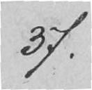37.
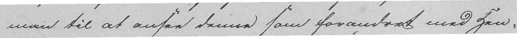man til at ansee denne som forandret med Hen-
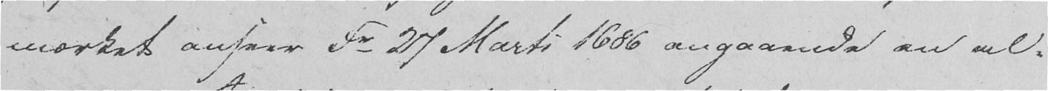mærket anseer Fr 27 Marts 1686 angaaende en al-
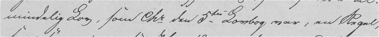mindelig Lov, som Chr den 5tes Lovbog var, en Regel,
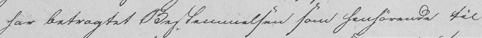har betragtet Bestemmelsen som henhørende til
